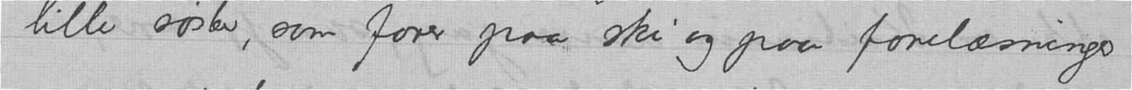lille søster, som farer paa ski og paa forelæsninger
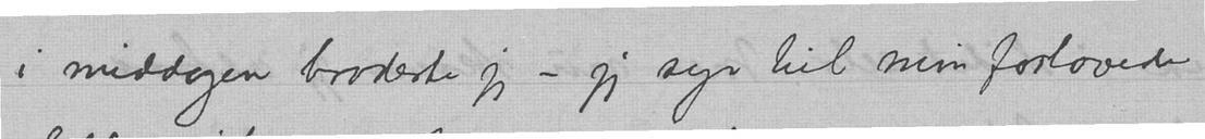i middagen broderte jeg - jeg syr til min forlovede
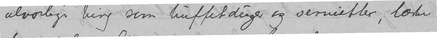alvorlige ting som buffetduger og servietter, læste
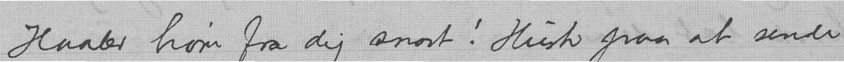Haaber høre fra dig snart! Husk paa at sende
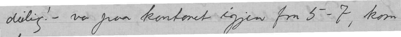deilig! - var paa kontoret igjen fra 5 - 7, kom
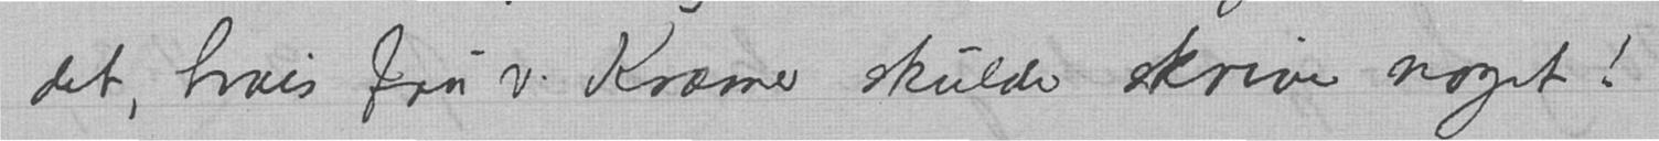det, hvis fru v. Kræmer skulde skrive noget!
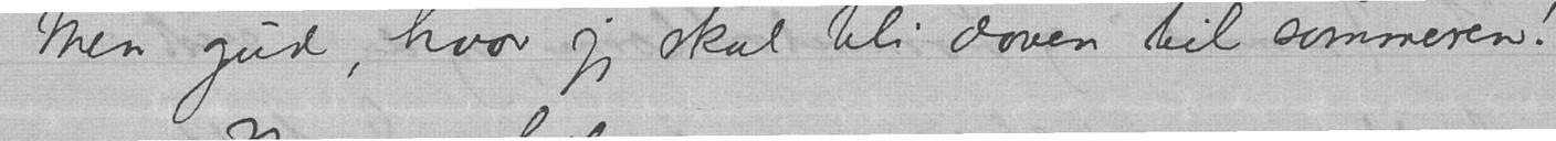Men gud, hvor jeg skal bli doven til sommeren!
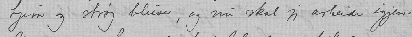hjem og strøg bluse, og nu skal jeg arbeide igjen.
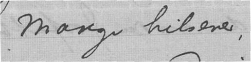Mange hilsener,
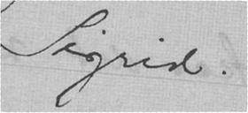Sigrid
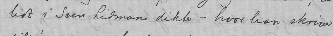lidt i Sven Lidmans dikter - hvor han skriver
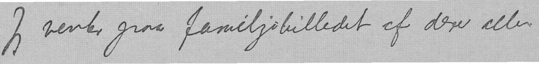Jeg venter paa familjebilledet af dere alle.
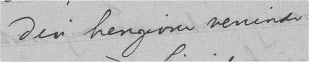Din hengivne veninde
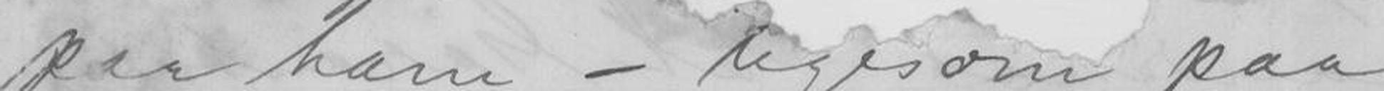paa ham - ligesom paa
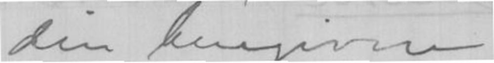Din hengivne
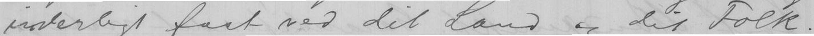inderlig fast ved dit Land og dit Folk.
